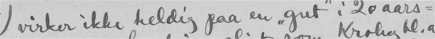virker ikke heldig paa en "gut" i 20 aars-
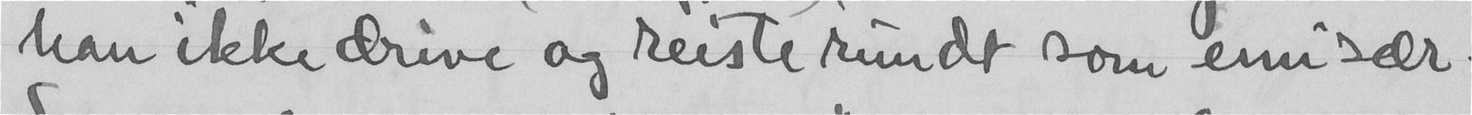han ikke drive og reiste rundt som enn sær.
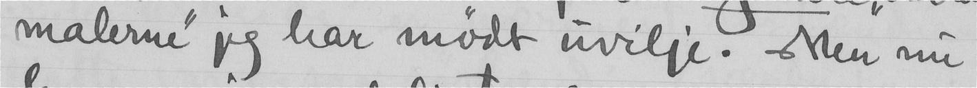malerne" jeg har mødt uvilje. Men nu
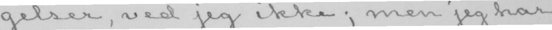gelser, ved jeg ikke; men jeg har
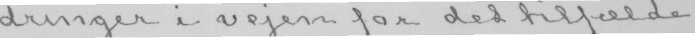dringer i vejen for det tilfælde
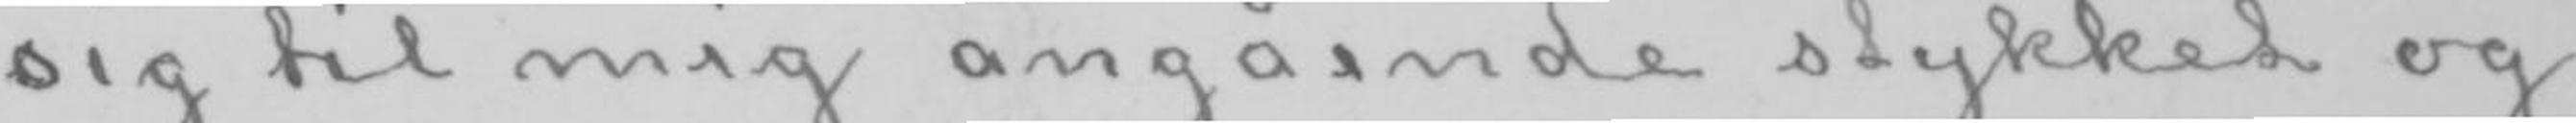sig til mig angående stykket og
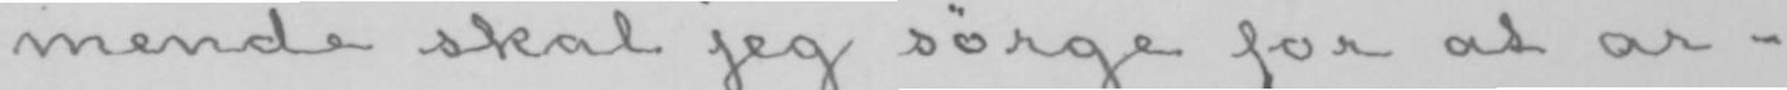mende skal jeg sørge for at ar-
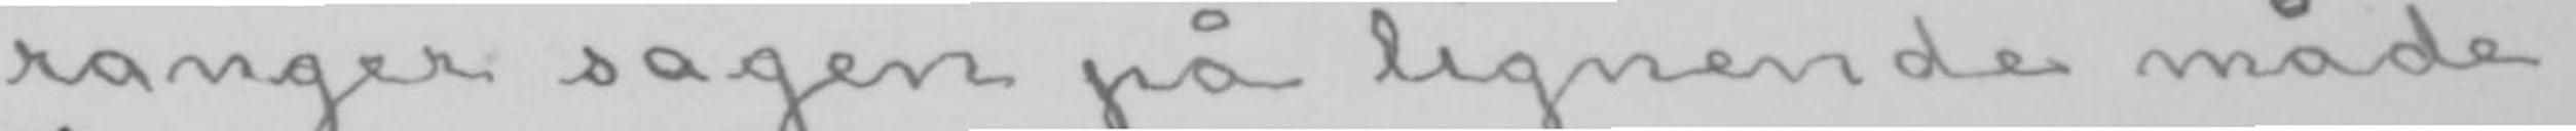rangere sagen på lignende måde.
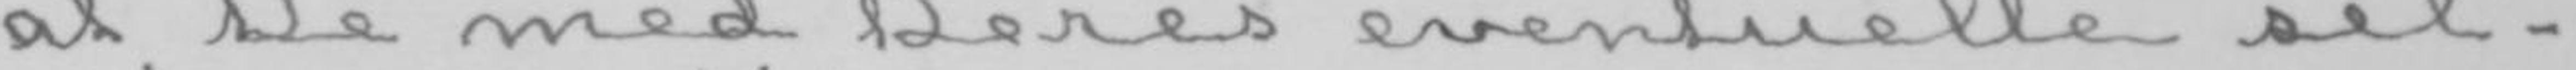at De med Deres eventuelle sel-
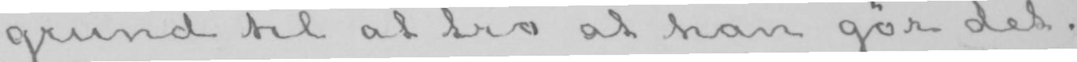grund til at tro at han gør det.
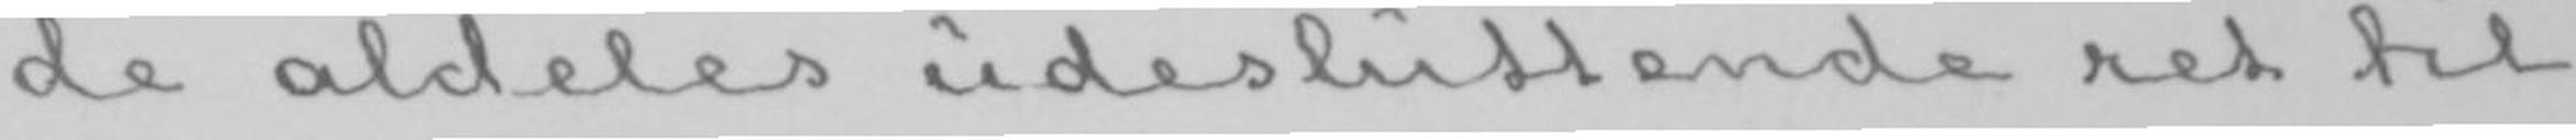de aldeles udesluttende ret til
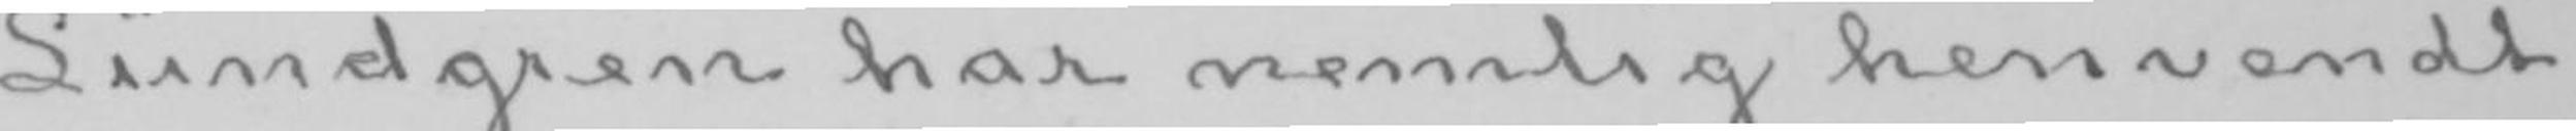Lundgren har nemlig henvendt
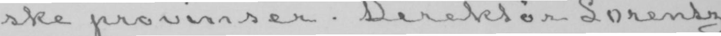ske provinser. Direktør Lorentz
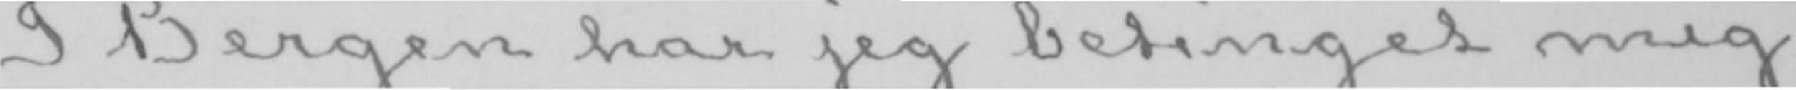I Bergen har jeg betinget mig
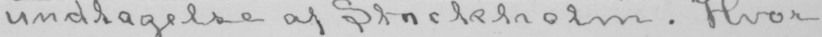undtagelse af Stockholm. Hvor
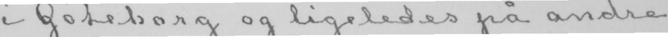i Göteborg og ligeledes på andre
